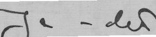Ja - det
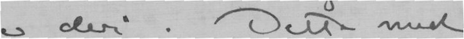er deri. Dette med
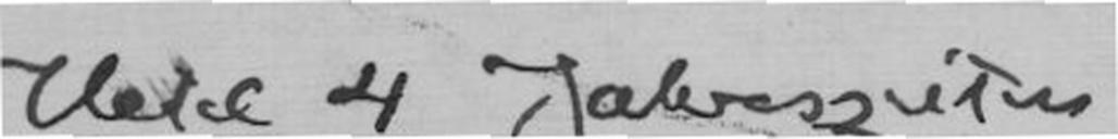Hotel 4 Jahressuiten
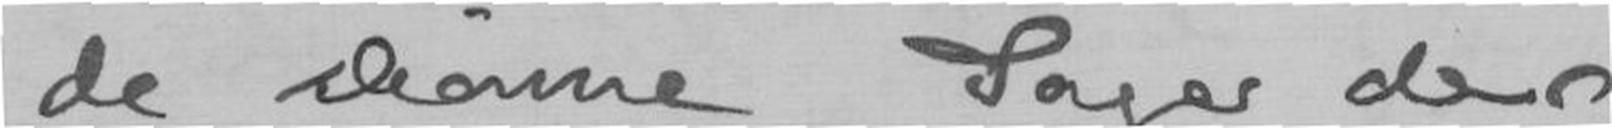de skønne Sager det
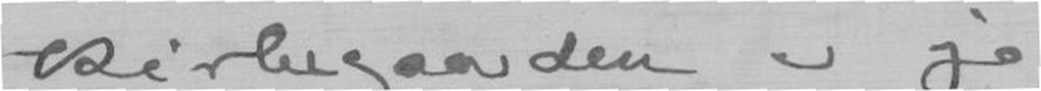Kirkegaarden er jo
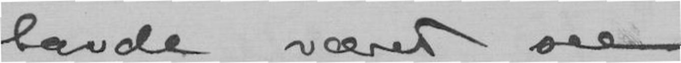havde været saa
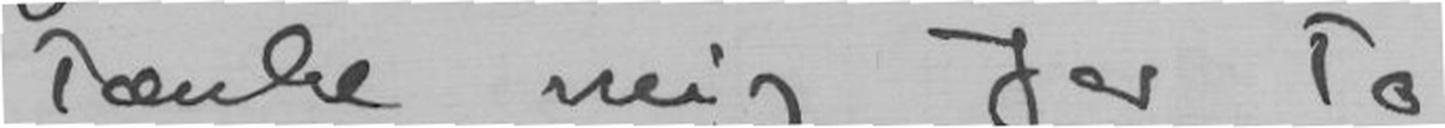tænke mig Jer to
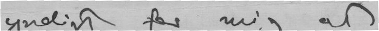yndigt for mig at
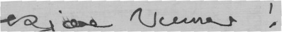Kjære Venner !
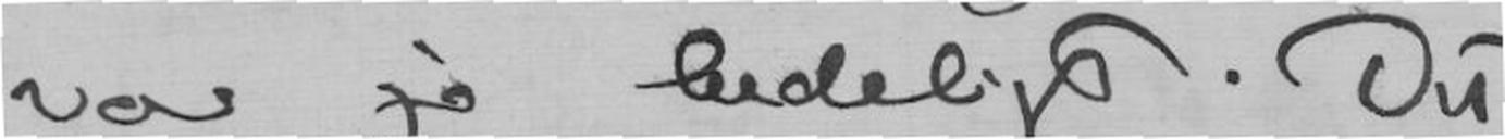var jo kedeligt. Det
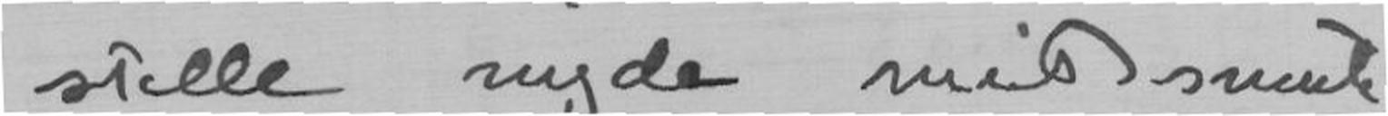stelle nyde mit smuk
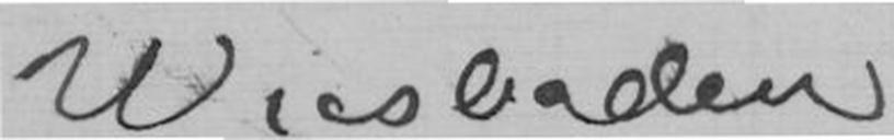Wiesbaden
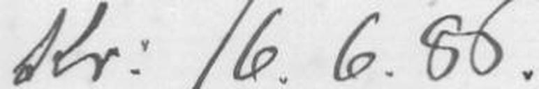Kr: 16.6.86
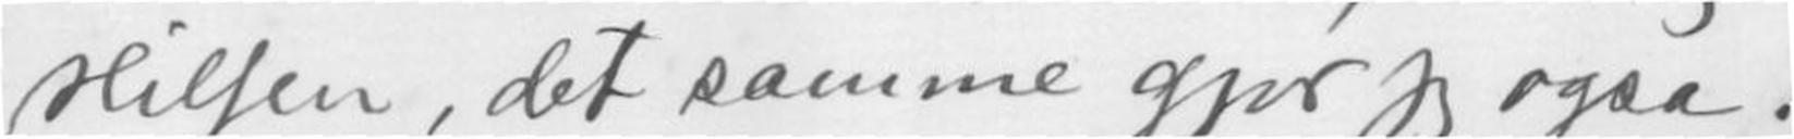Hilsen, det samme gjør jeg ogsa.
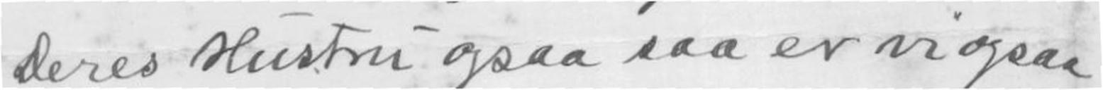Deres Hustru ogsaa saa er vi ogsaa
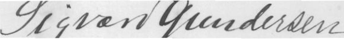Sigvard Gundersen
